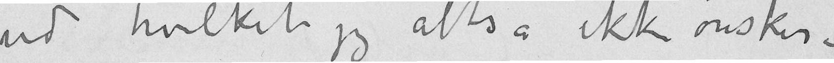ud hvilket jeg altså ikke ønsker –
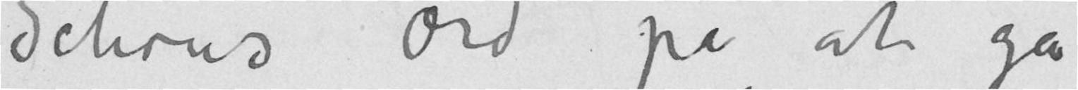Schous ord på at gå
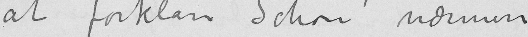at forklare Schou nærmere
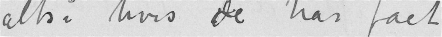altså hvis De har fået
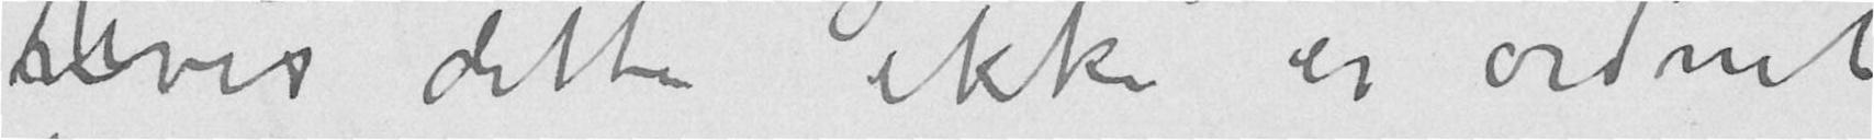hvis dette ikke er ordnet
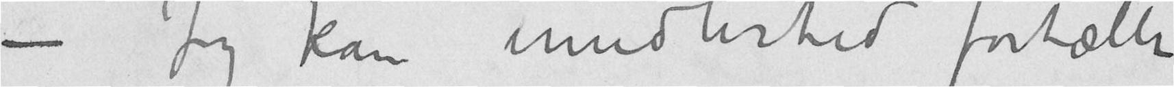– Jeg kan imidlertid fortælle
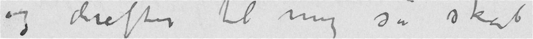og derefter til mig så skal
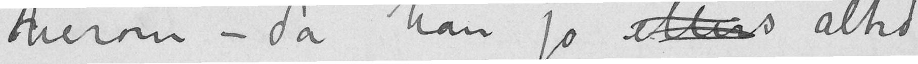herom – da han jo ellers altid
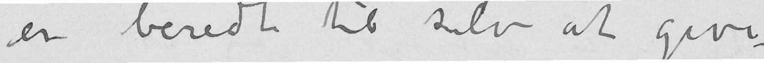er beredt til selv at give
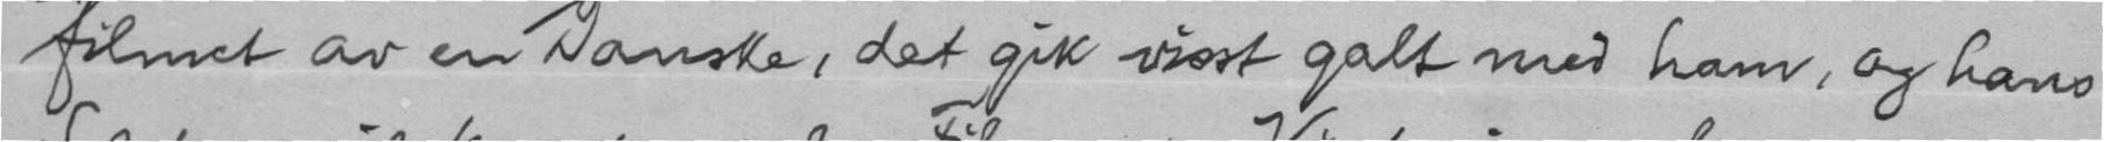filmet av en Danske, det gik visst galt med ham, og hans
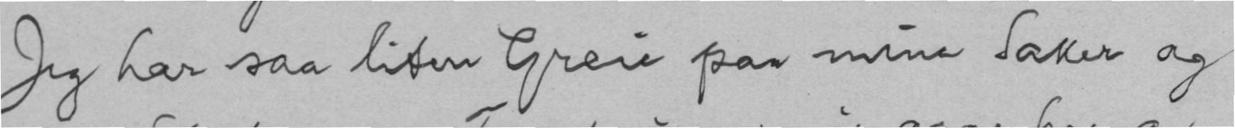Jeg har saa liten Greie paa mine Saker og
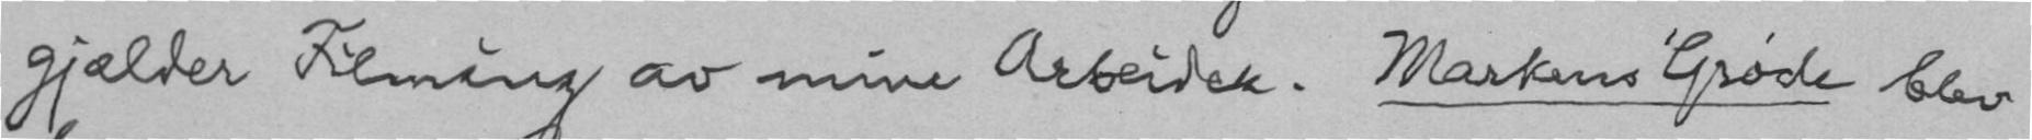gjælder Filming av mine Arbeider. Markens Grøde blev
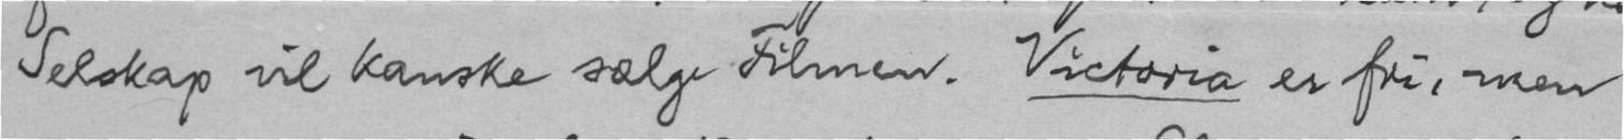Selskap vil kanske sælge Filmen. Victoria er fri, men
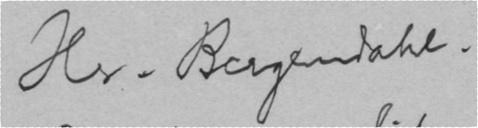Hr. Bergendahl.
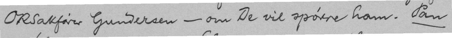ORSakfører Gundersen - om De vil spørre ham. Pan
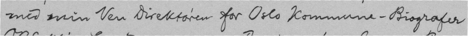med min Ven Direktøren for Oslo Kommune-Biografer
